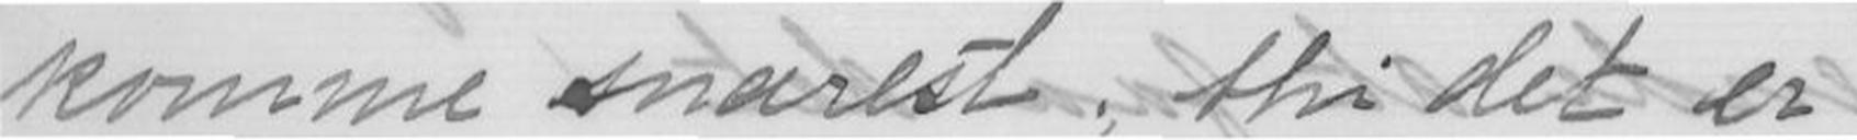komme snarest; thi det er
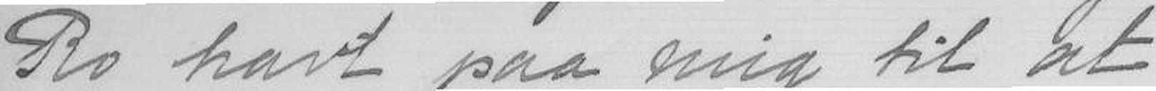Ro havt paa mig til at
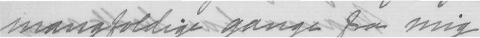mangfoldige ganger fra mig
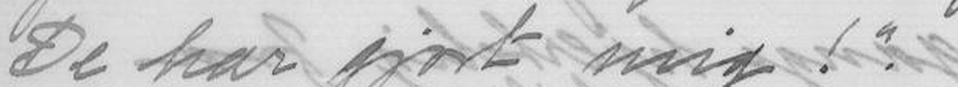De har gjort mig!"
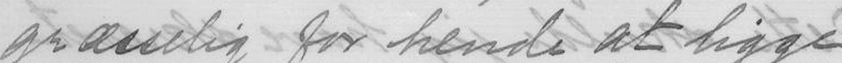græulig for hende at ligge
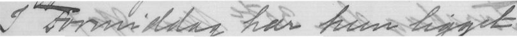I Formiddag har hun ligget
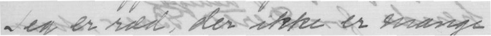Jeg er ræd, der ikke er mange
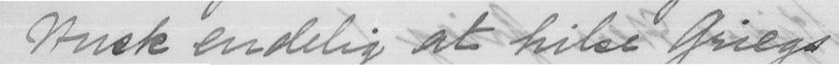"Husk endelig at hilse Griegs
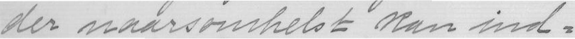der naarsomhelst man ind-
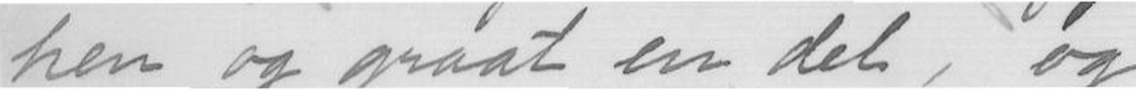her og graat en del, og
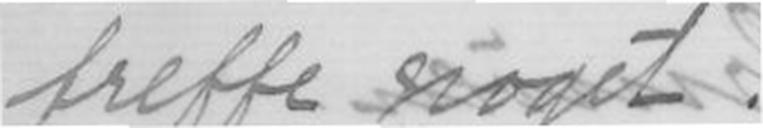treffe noget.
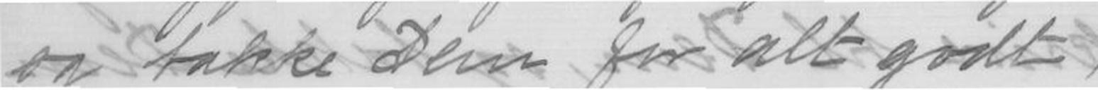og takke Dem for alt godt,
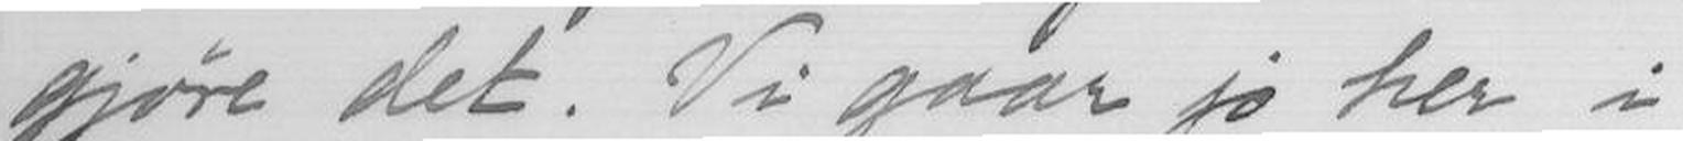gjøre det. Vi gaar jo her i
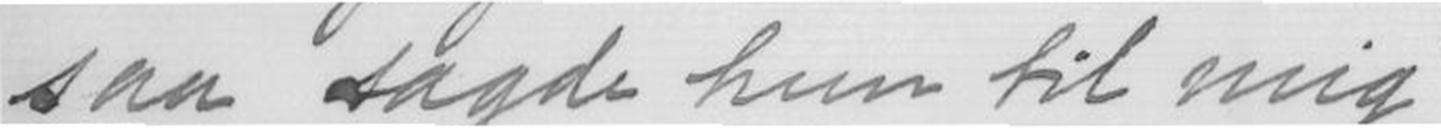saa tagde hun til mig:
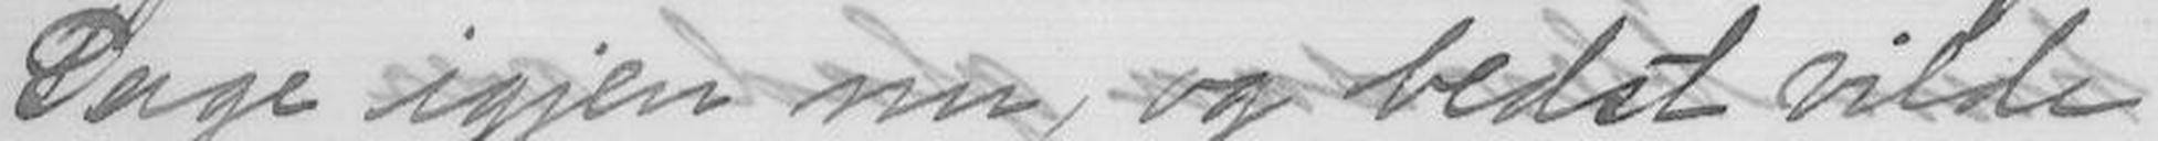Dage igjen nu, og bedst vilde
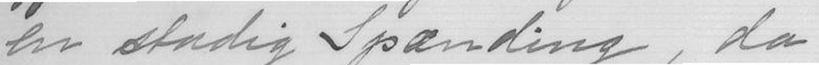en stadig Spænding, da
